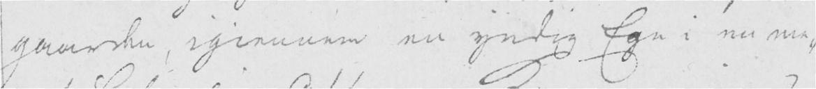gaarden, igiennem en yndig Egn i en me-
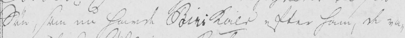Søn, som nu havde Biri Kald efter ham, de va-
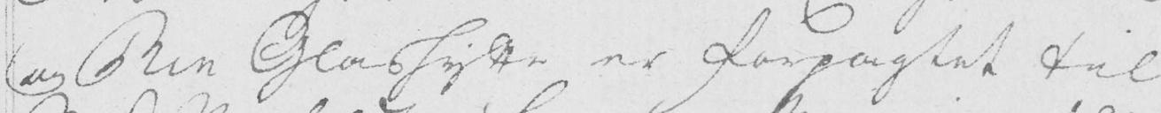og Biri Glashytte er forpagtet til
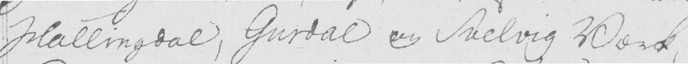Hallingdal, Hurdal og Svelvig Værk,
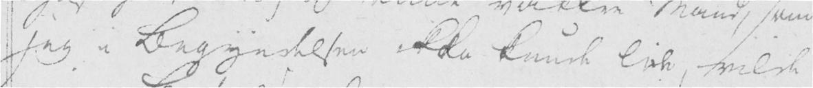jeg i Begyndelsen ikke kunde lide, vilde
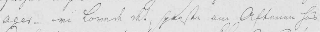ager - vi lovede det, spiste om Aftenen hos
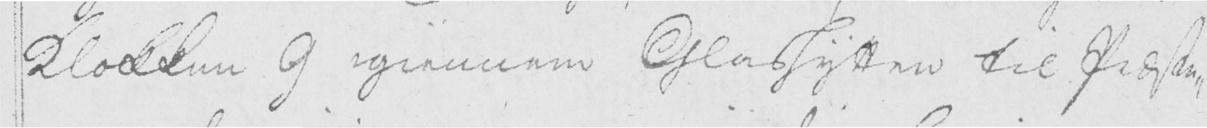Klokken 9 igiennem Glashytten til Præste-
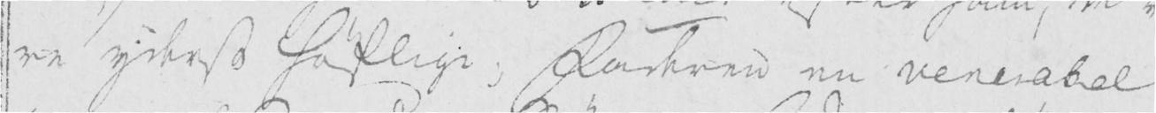re yderst høflige; Faderen en venerabel
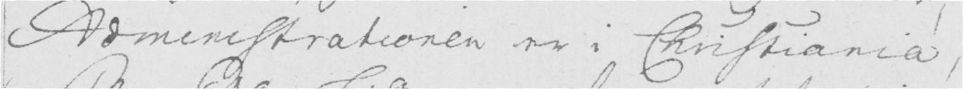Administrationen er i Christiania,
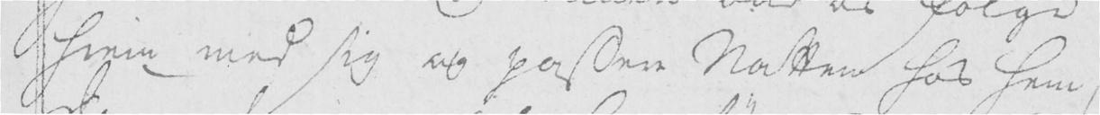hiem med sig og passere Natten hos ham,
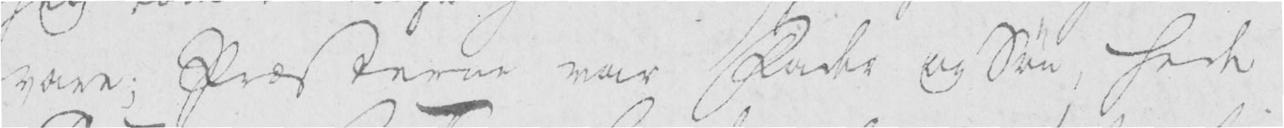vare; Præsterne var Fader og Søn, hede
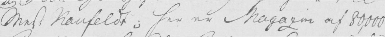Mest Kaufeldt; her er Magazin af 80,000
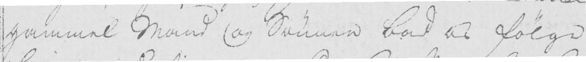gammel Mand og Sønnen bad os følge
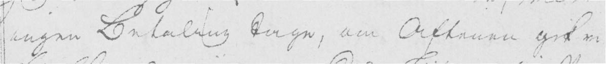ingen Betaling tage, om Aftenen gik vi
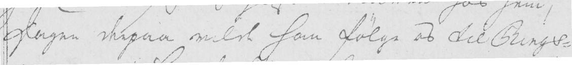Dagen derpaa vilde han følge os til Rings-
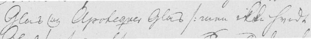Glas og Apotequer Glas /: men ikke hvidt
